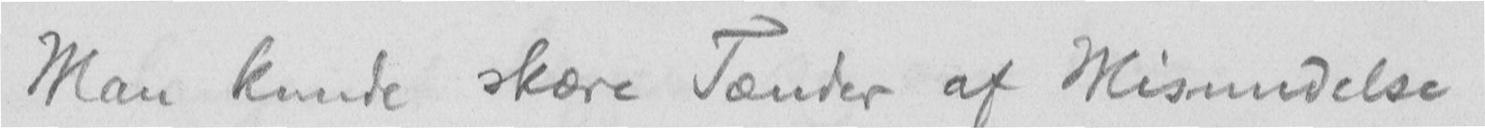Man kunde skære Tænder af Misundelse
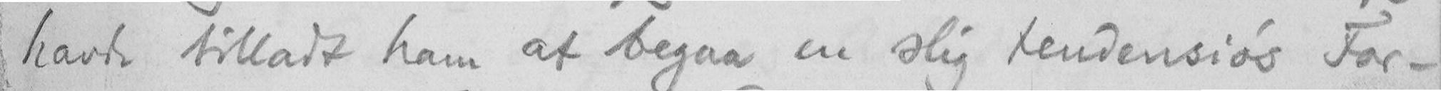havde tilladt ham at begaa en slig tendensiøs For-
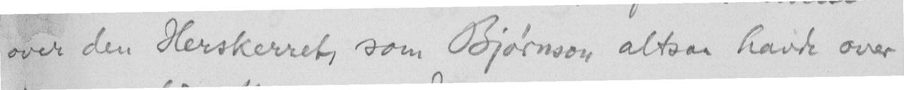over den Herskerret, som Bjørnson altsaa havde over
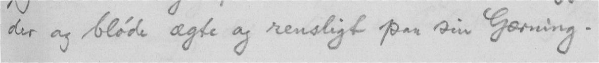der og bløde ægte og rensligt paa sin Gærning.
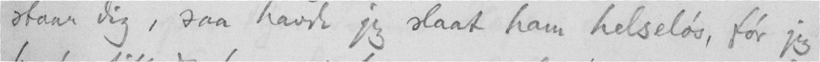staar dig, saa havde jeg slaat ham helseløs, før jeg
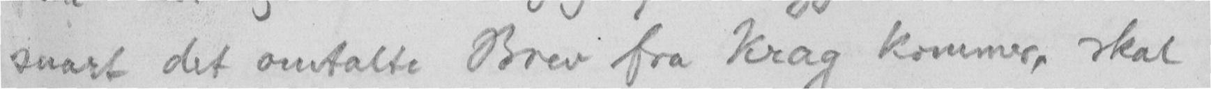snart det omtalte Brev fra Krag kommer, skal
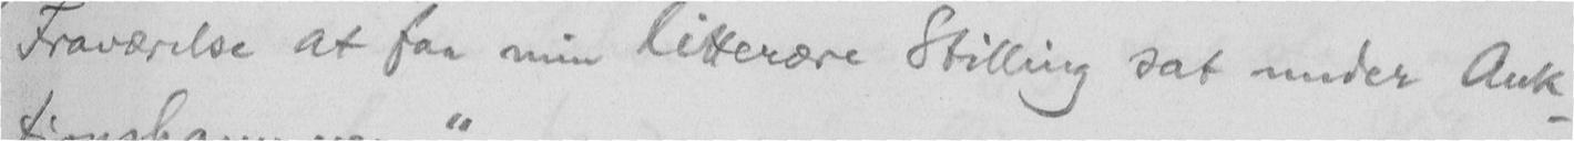Fraværelse at faa min litterære Stilling sat under Auk-
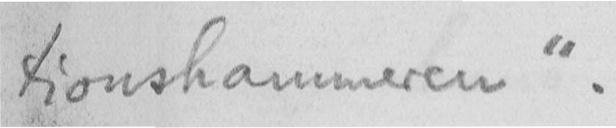tionshammeren".
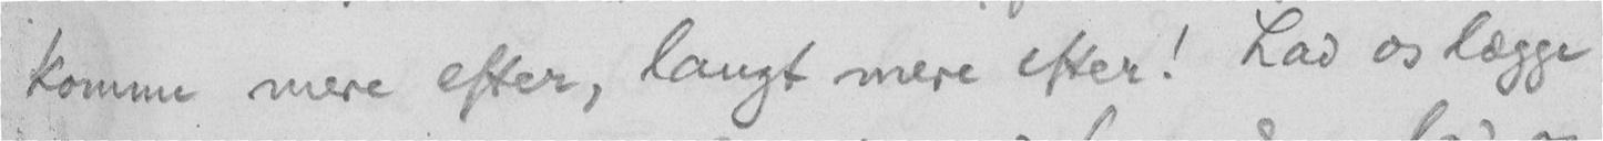komme mere efter, langt mere efter! Lad os lægge
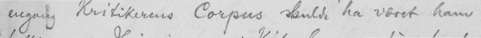engang Kritikerens Corpus skulde ha været ham
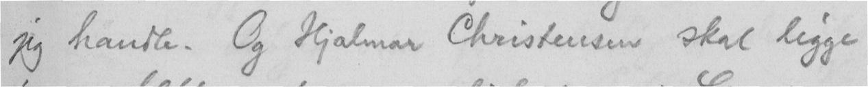jeg handle. Og Hjalmar Christensen skal ligge
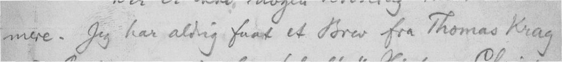mere. Jeg har aldrig faat et Brev fra Thomas Krag
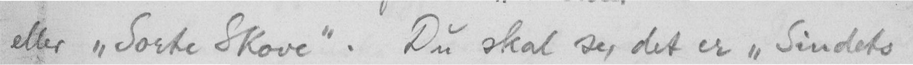eller "Sorte Skove". Du skal se, det er "Sindets
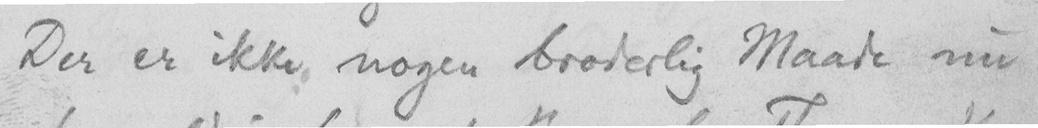Der er ikke nogen broderlig Maade nu
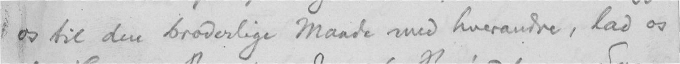os til den broderlige Maade med hverandre, lad os
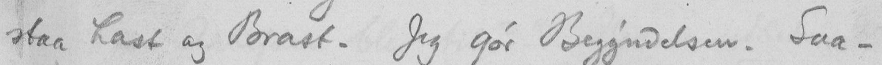staa Last og Brast. Jeg gør Begyndelsen. Saa-
